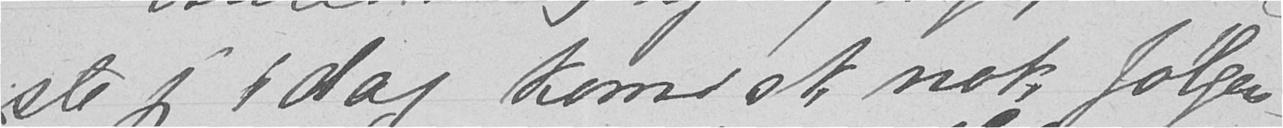ste jeg idag komisk nok følgen-
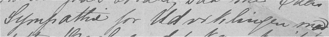Sympathie for Udviklingen med
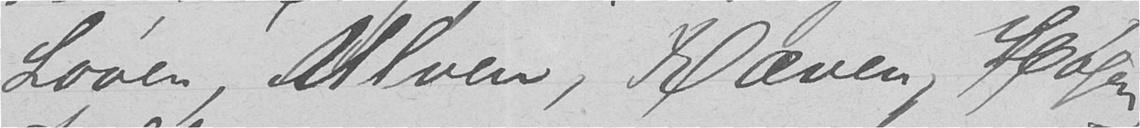Løven, Ulven, Ræven Høgen
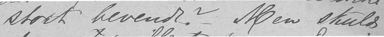stort bevendt? Men skulde
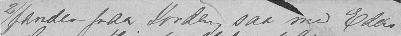2) findes paa Jorden, saa med Eders
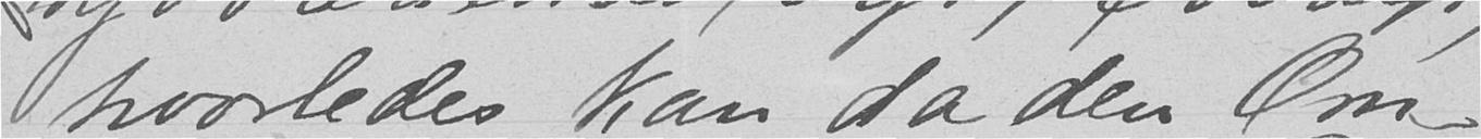hvorledes kan da den Om-
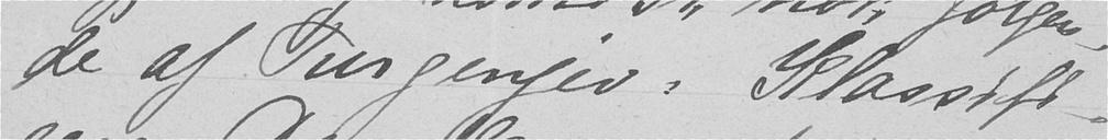de af Turgenjev: Klassifi-
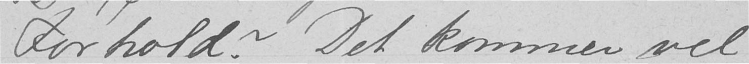Forhold? Det kommer vel
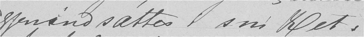gjenindsættes i sin Ret.
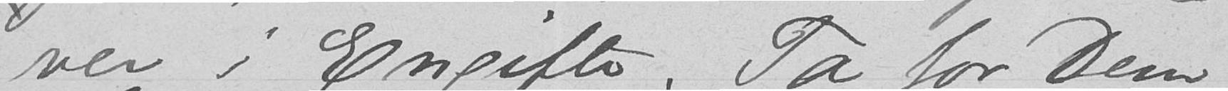ver i Engifte. Ta for Dem
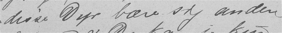disse Dyr bære sig ander-
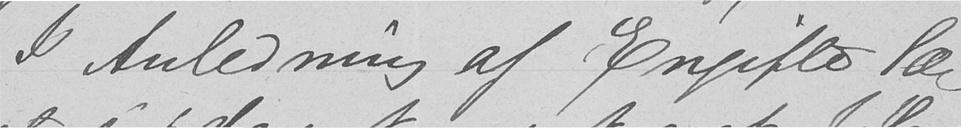I Anledning af Engifte læ-
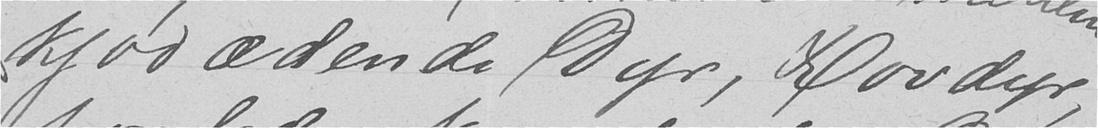kjødædende Dyr, Rovdyr,
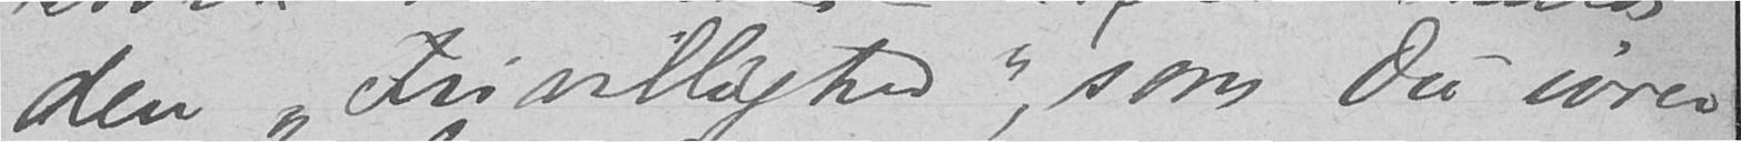den "Frivillighed", som Du ivrer
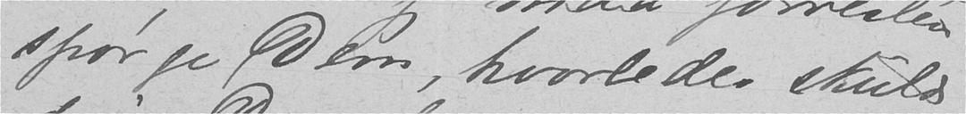spørge Dem, hvorledes skulde
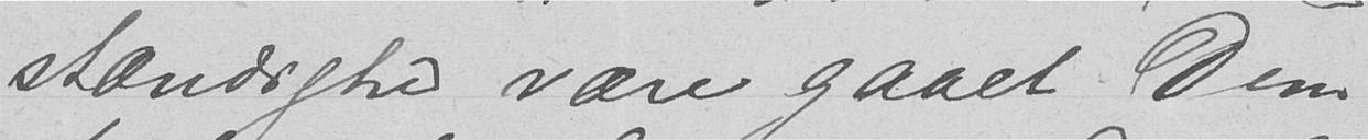stændighed være gaaet Dem
